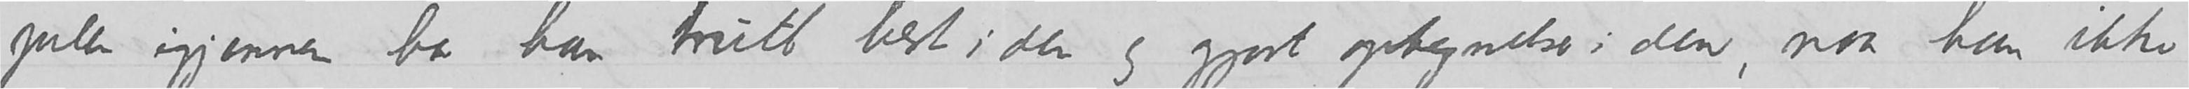julen igjennem har han trutt lest i den og gjort optegnelser i den, naar han ikke
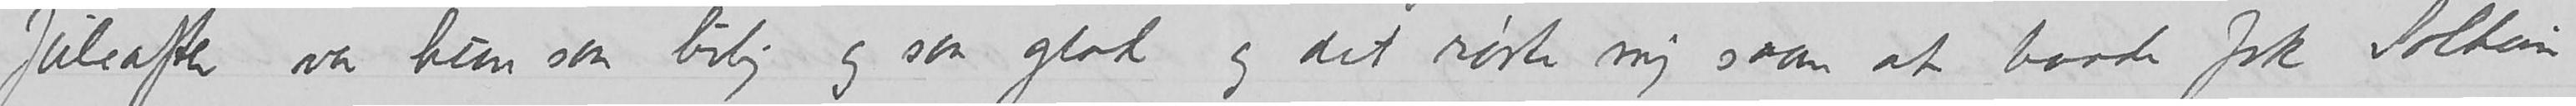Juleaften var hun saa livlig og saa glad og det rørte mig saan at baade frk Solheim
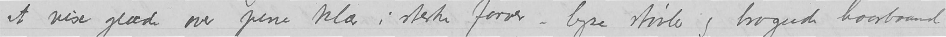at vise glæde over pene klær i sterke farver – lyse støvler og brogede haarbaand
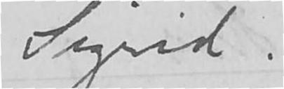Sigrid.
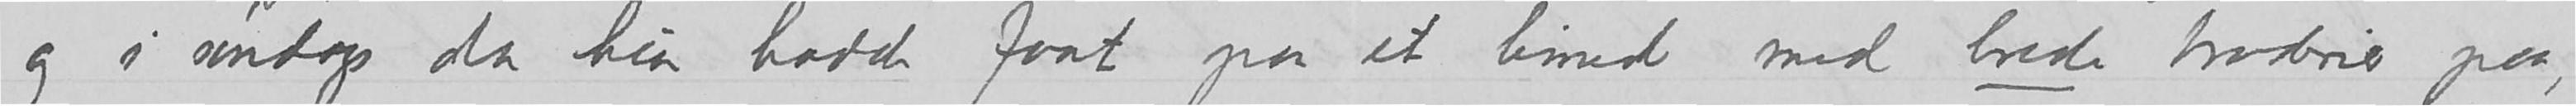og i søndags da hun hadde faat paa et linned med brede brodrier paa,
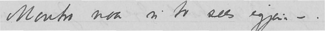Montro naar vi to sees igjen. –
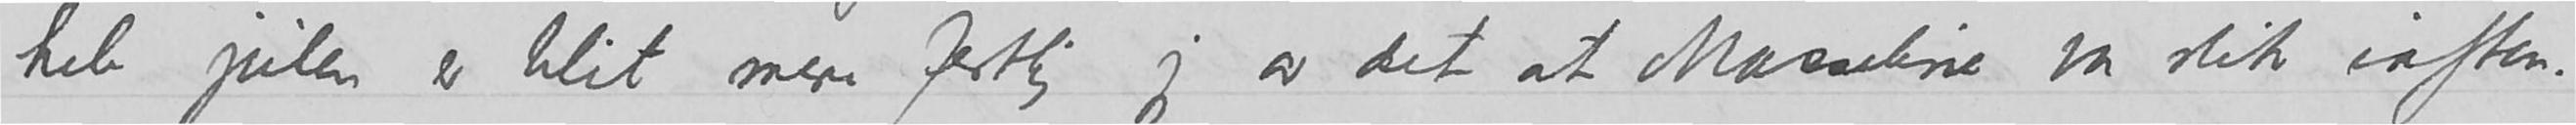hele julen er blit mere festlig jeg av det at Mosseline var slik iaften.»
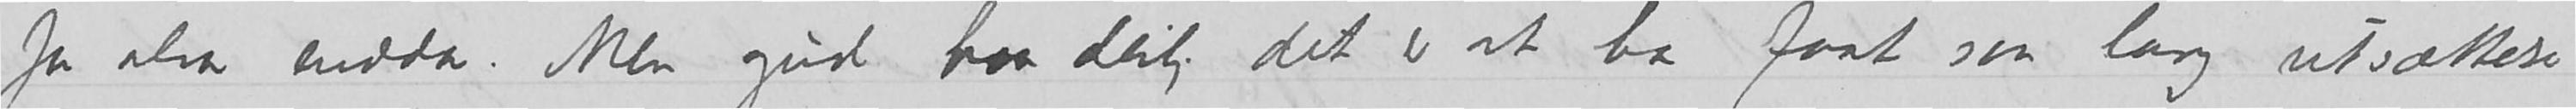for alvor endda.  Men gud hvor deilig det er at ha faat saa lang utsættelse
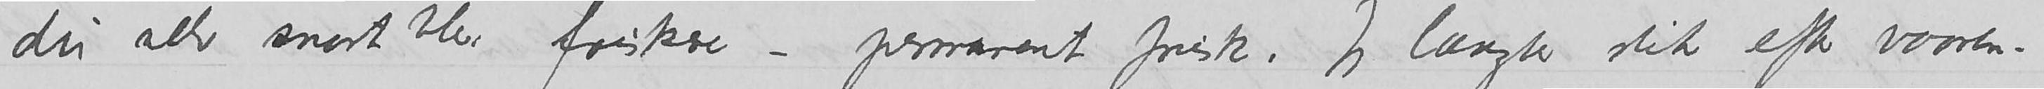du selv snart blev friskere – permanent frisk.  Jeg længter slik efter vaaren -
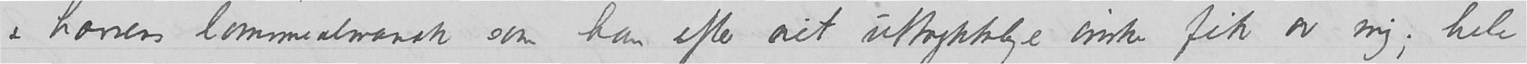& Larsens lommealmanak som han efter sit uttrykkelige ønske fik av mig; hele
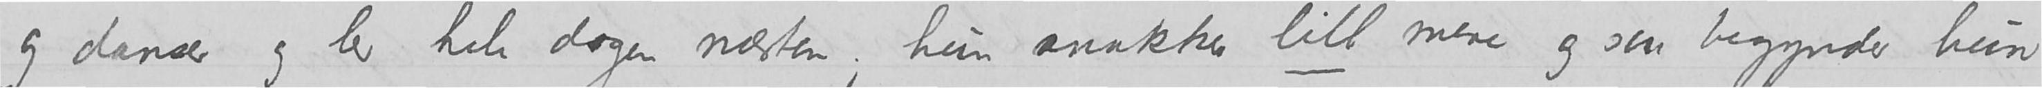og danser og ler hele dagen næsten; hun snakker litt mere og saa begynder hun
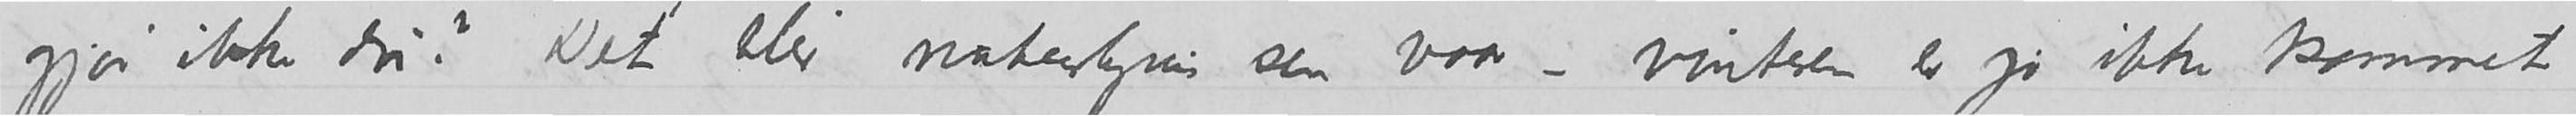gjør ikke du?  Det blir naturligvis sen vaar – vinteren er jo ikke kommet
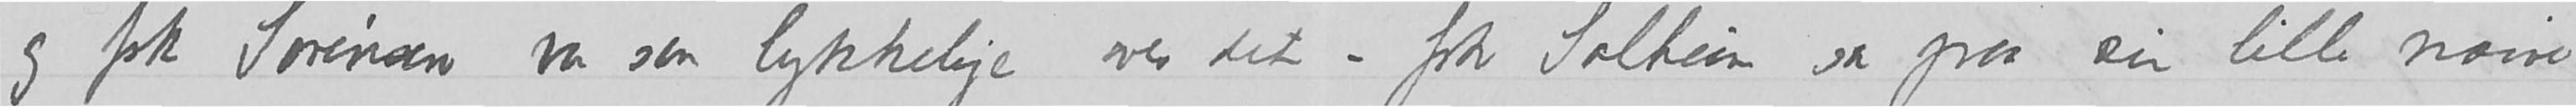og frk Sørensen var saa lykkelige over det – frk Solheim sa paa sin lille naive
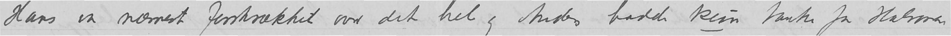Hans var nærmest forskrækket over det hele og Anders hadde kun tanke for Halvorsens
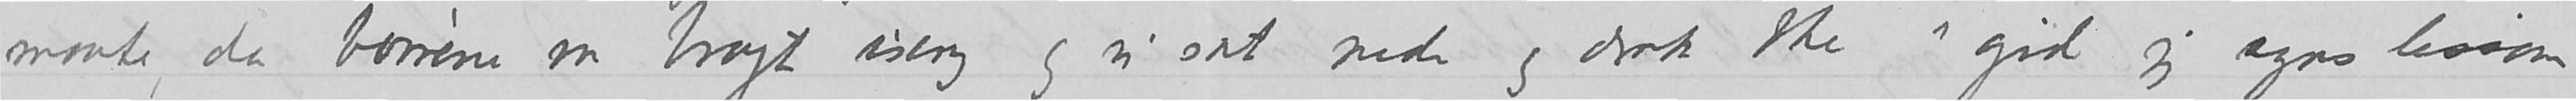maate, da børnene var bragt iseng og vi sat nede og drak the  «gid jeg syns lissom
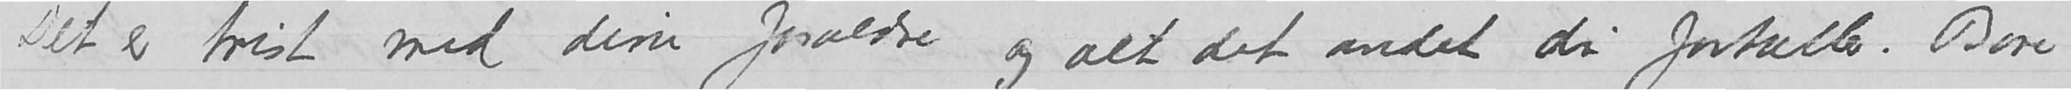Det er trist med dine forældre og alt det andet du fortalte.  Bare
